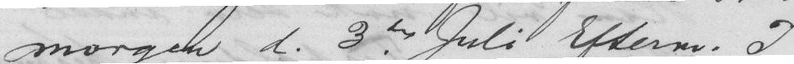morgen d. 3die Juli Efterm. I
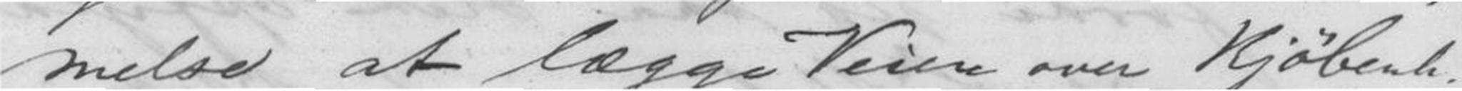melse at lægge Veien over Kjøbenh.
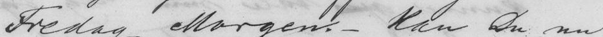Fredag Morgen. - Kan Du nu
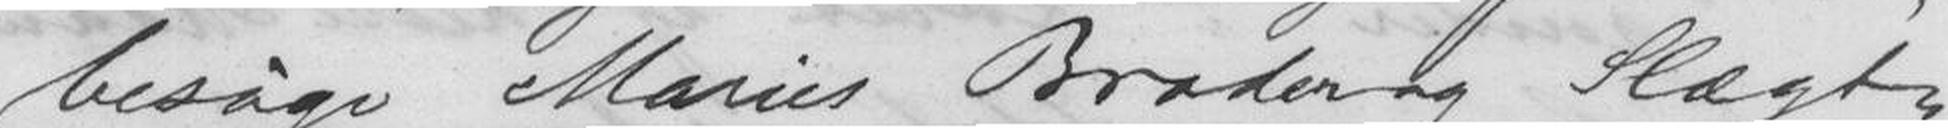besøge Maries Broder og Slægt-
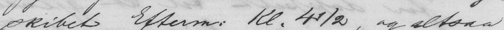skibet, Efterm. Kl.4½, og altsaa
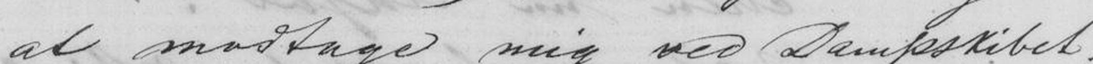at modtage mig ved Dampskibet;
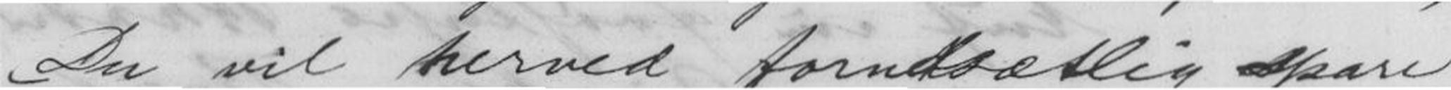Du vil herved forudsætlig spare
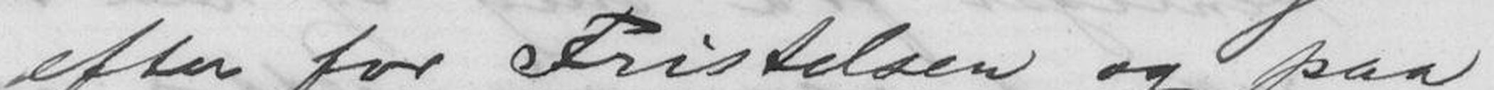efter for Fristelsen og paa
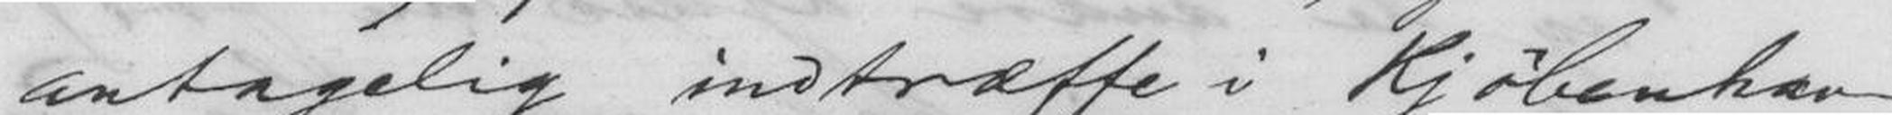antagelig indtræffe i Kjøbenhavn
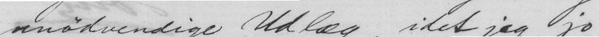unødvendige Udlæg, idet jeg jo
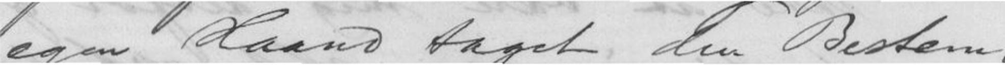egen Haand taget den Bestem-
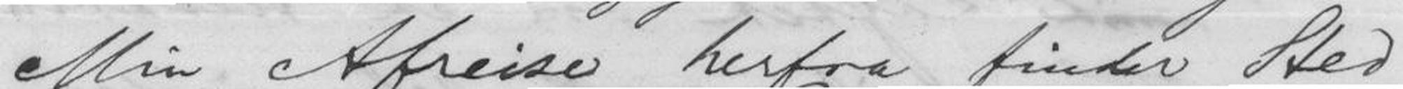Min Afreise herfra finder Sted
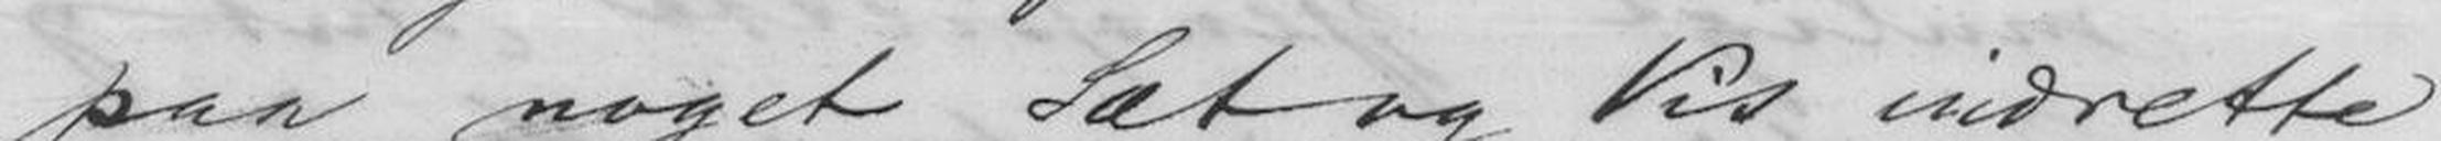paa noget Sæt og Vis indrette
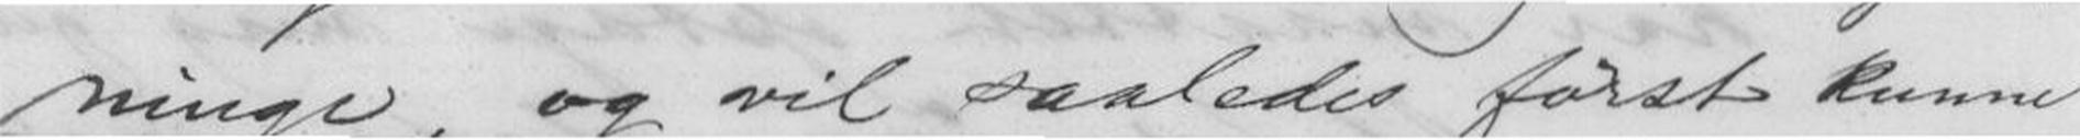ninge, og vil saaledes først kunne
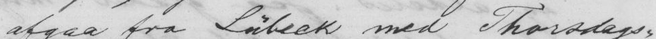afgaa fra Lübeck med Thorsdags-
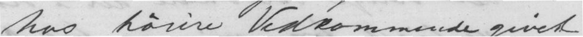hos høiere Vedkommende givet
